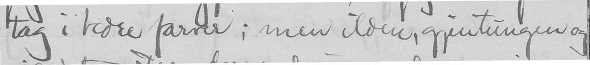tag i bedre farver; men ilden, gjentungen og
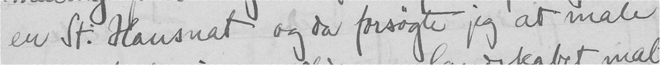en St. Hansnat og da forsøgte jeg at male
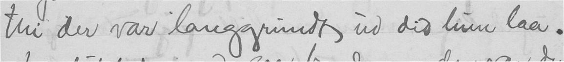thi der var langgrundt, ud did hun laa.
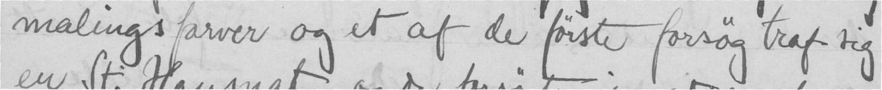malingsfarver og et af de første forsøg traf sig
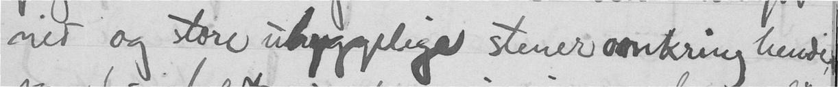ned og store uhyggelige stener omkring hende
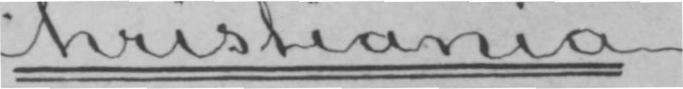Christiania.
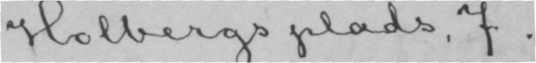Holbergs plads, 7.
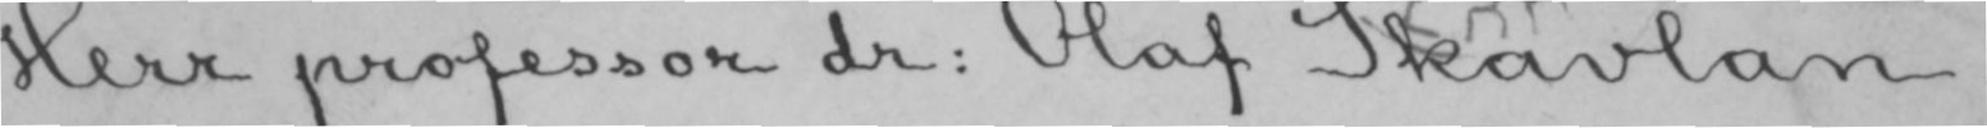Herr professor dr: Olaf Skavlan.
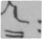fr.
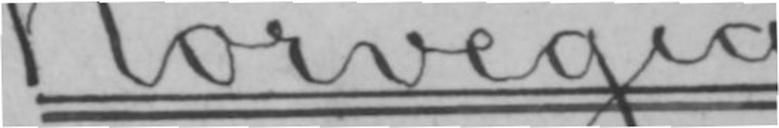(Norvegia.)
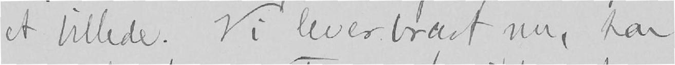et billede. Vi lever bravt nu, har
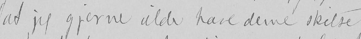at jeg gjerne vilde have denne skitse
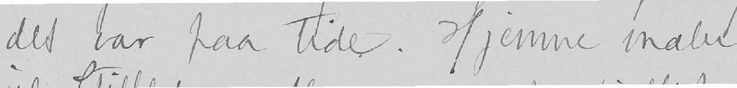det var paa tide. Hjemme maler
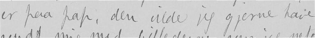er paa pap, den vilde jeg gjerne have
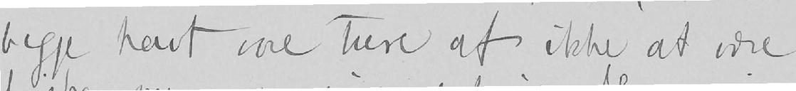begge havt vore ture af ikke at være
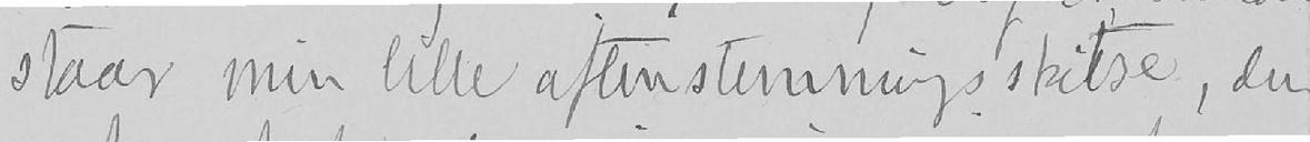staar min lille aftenstemningsskitse, der
